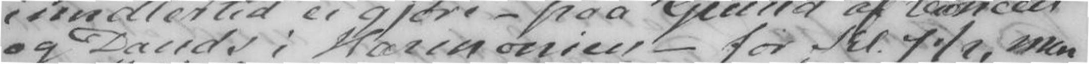og Dands i Harmonien - for Kl 7½, med
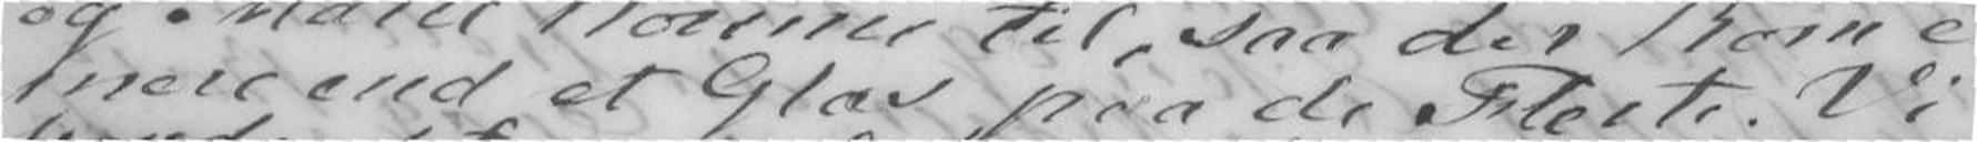mere end et Glas paa de Fleste. Vi
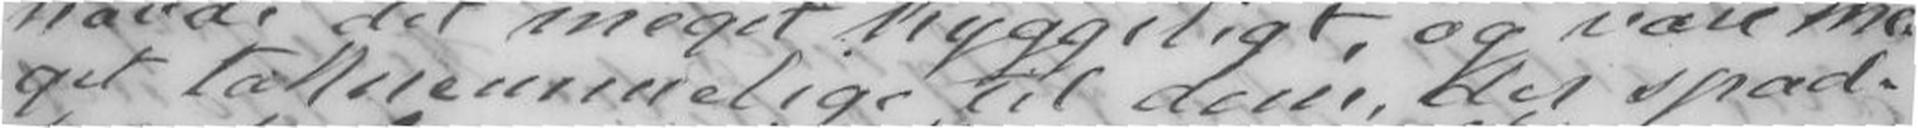get taknemmelige til dem, der spad-
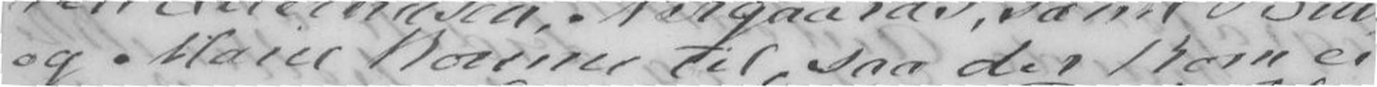og Marie komne til, saa der kom ei
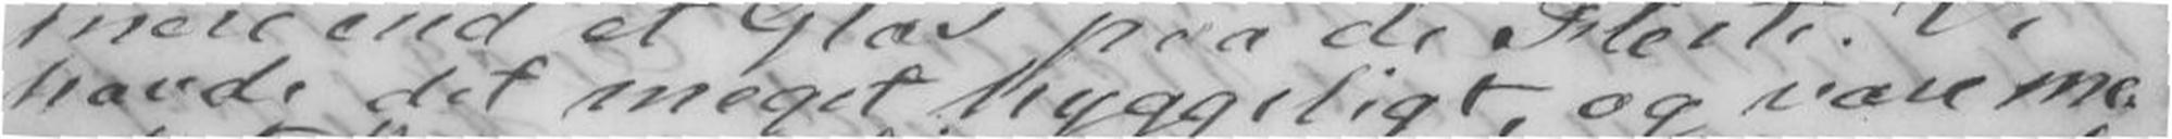havde det meget hyggeligt, og vare me-
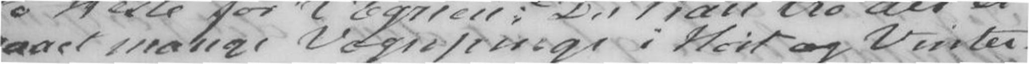gaaet mange Vognpenge i Høst og vinter.
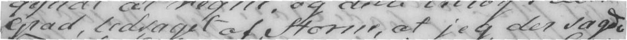Grad, ledsaget af Storm, at jeg der sagde
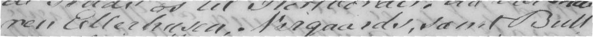ren Ellerhusen, Nergaards, samt Bull
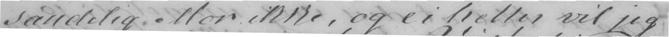sandelig Mor ikke, og ei heller vil jeg
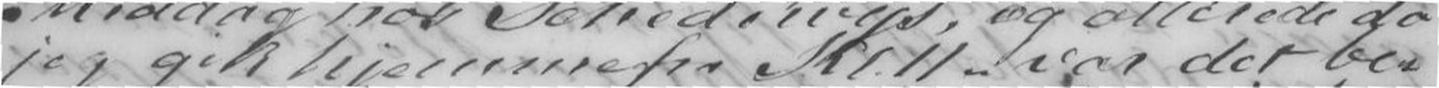jeg gik hjemmefra Kl.11 - var det be-
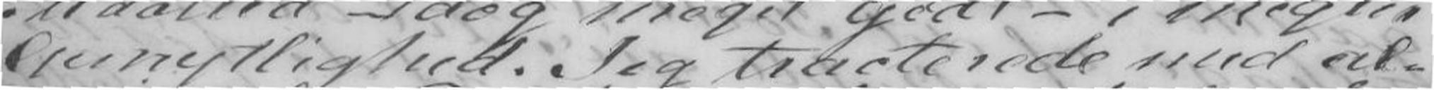Gemytlighed. Jeg tracterede med al-
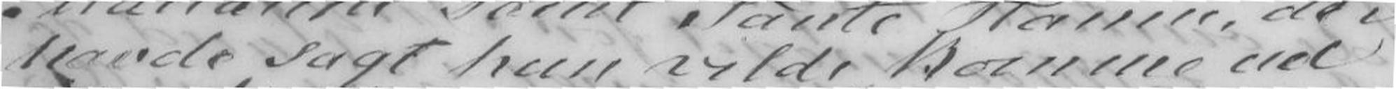havde sagt hun vilde komme ud
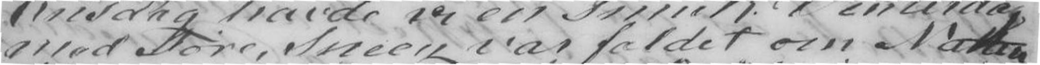med Tøre, Sneen var faldet om Natten
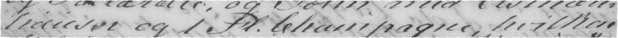hairser og 1 Fl Champagne, hvilken
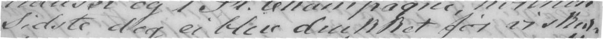sidste dog ei blev drukket før vi skul-
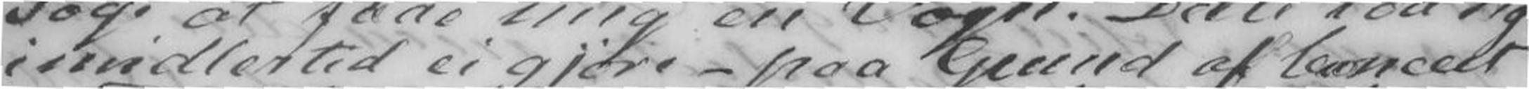imidlertid ei gjørre - paa Grund af Concert
